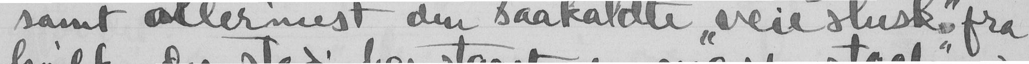samt allermest den saakaldte "veieslusk" fra
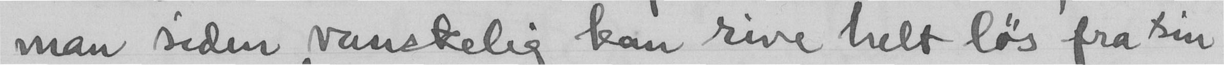man siden vanskelig kan rive helt løs fra sin
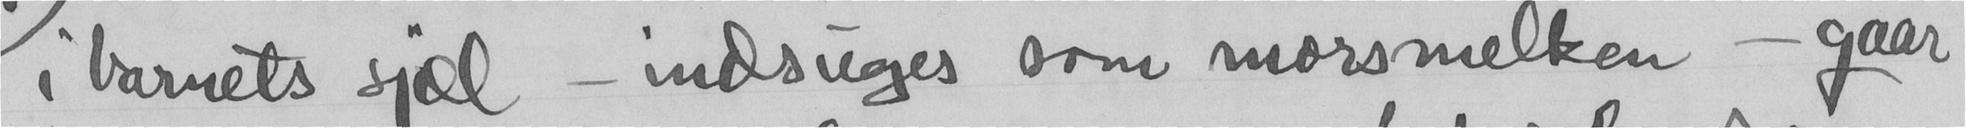i barnets sjæl - indsuges som morsmelken - gaar
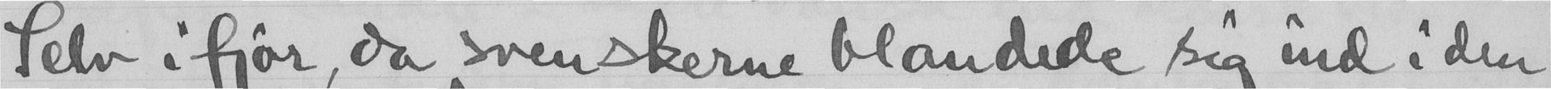Selv i fjor, da svenskerne blandede sig ind i den
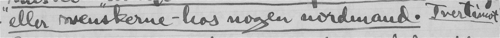eller svenskerne - hos nogen nordmand. Tvertimot
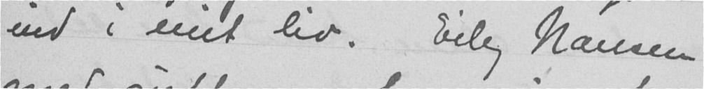ind i mit liv. Eileg Nansen
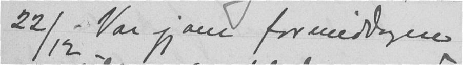22/12 Var jeg ane formiddagen
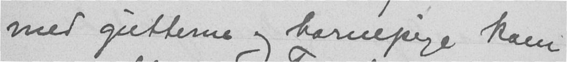med gutterne og barnepige kom
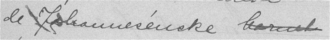de Johannesénske barnet
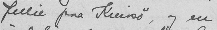Julie paa Knivsø og en
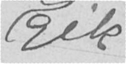gik
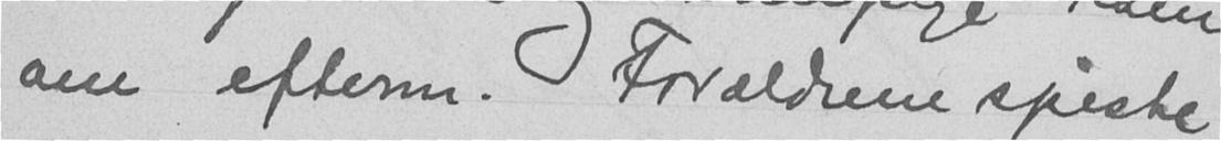om efterm. Forældrene spiste
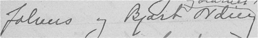Johans og Bjart Ording
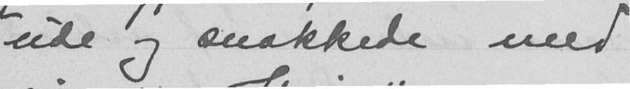ude og snakkede med
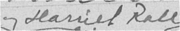og Harriet Roll
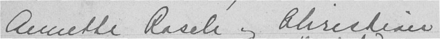Annette Rasch og Christian
